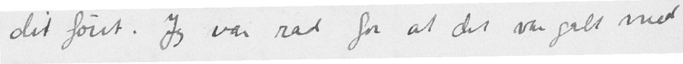dit først. Jeg var ræd for at det var galt med
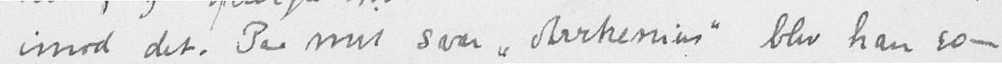imod det. Paa mit svar "Arrhenius" blev han som
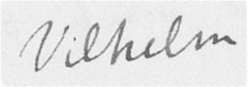Vilhelm
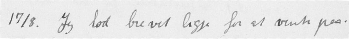17/8. Jeg lod brevet ligge for at vente paa
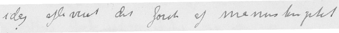i dag afleveret det første af manuskriptet
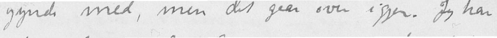gynde med, men det gaar over igjen. Jeg har
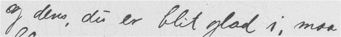og dens, du er blit glad i, maa
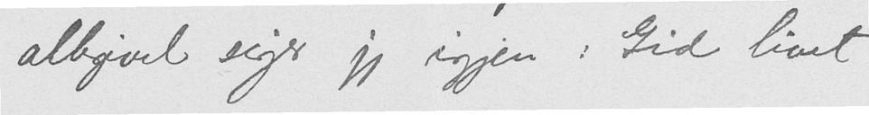alligevel siger jeg igjen: Gid livet
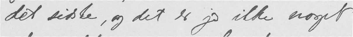det sidste, og det er jo ikke noget
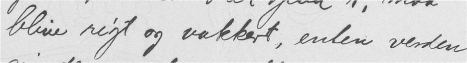blive rigt og vakkert, enten verden
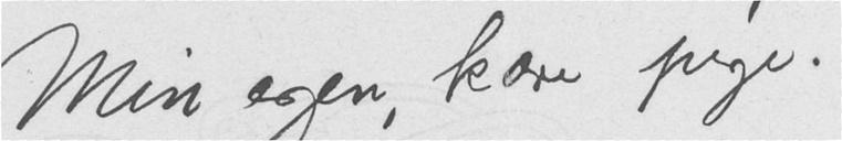Min egen, kære pige!
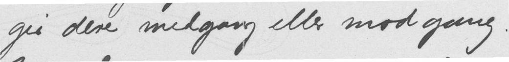gir dere medgang eller modgang.
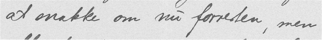at snakke om nu forresten, men
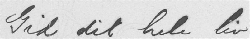Gid dit hele liv
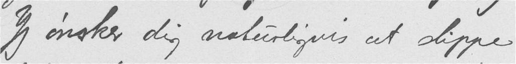Jeg ønsker deg naturligvis at slippe
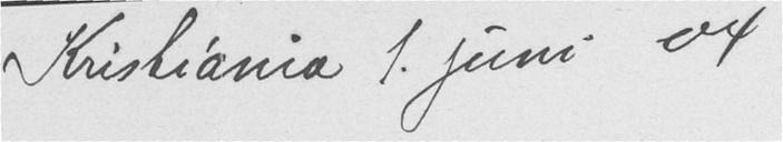Kristiania 1. juni 04
